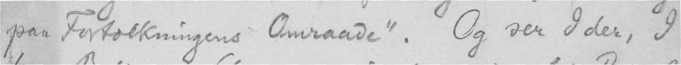paa Fortolkningens Omraade". Og ser I der, I
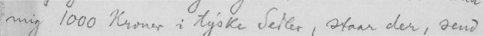mig 1000 Kroner i tyske Sedler, staar der, send
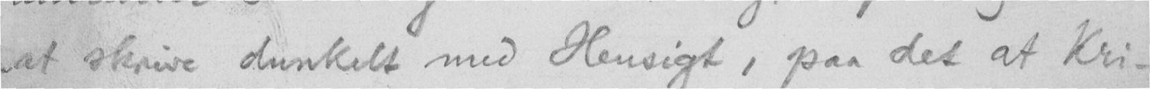at skrive dunkelt med Hensigt, paa det at Kri-
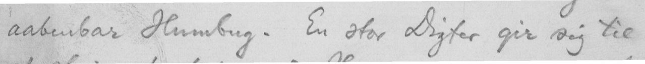aabenbar Humbug. En stor Digter gir sig til
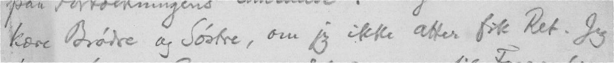kære Brødre og Søstre, om jeg ikke atter fik Ret. Jeg
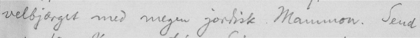velbjærget med megen jordisk Mammon. Send
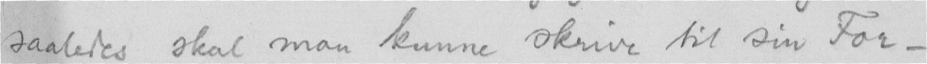saaledes skal man kunne skrive til sin For-
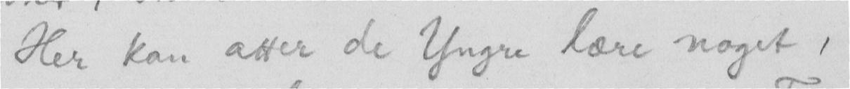Her kan atter de Yngre lære noget,
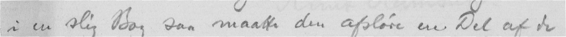i en slig Bog saa maatte den afsløre en Del af de
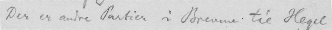Der er andre Partier i Brevene til Hegel
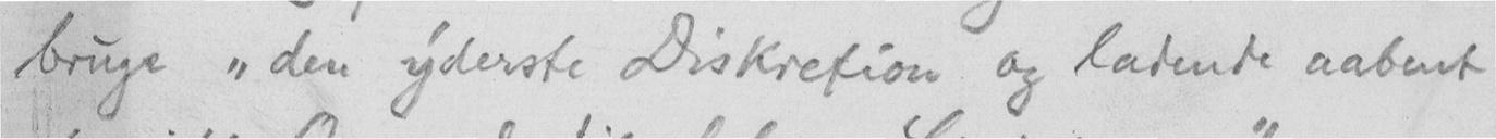bruge "den yderste Diskretion og ladende aabent
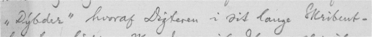"Dybder" hvoraf Digteren i sit lange Skribent-
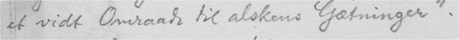et vidt Omraade til alskens Gætninger".
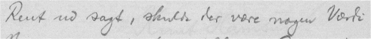Rent ud sagt, skulde der være nogen Værdi
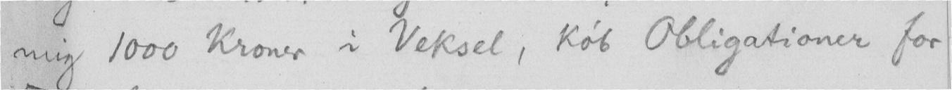mig 1000 Kroner i Veksel, køb Obligationer for
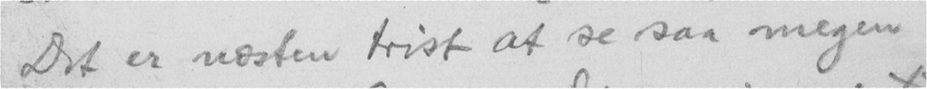Det er næsten trist at se saa megen
